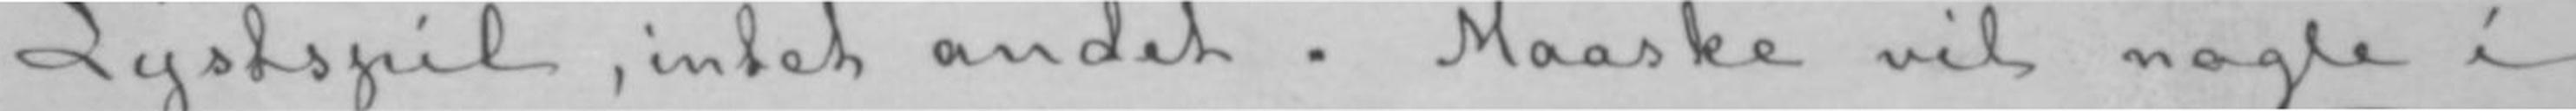Lystspil, intet andet. Maaske vil nogle i
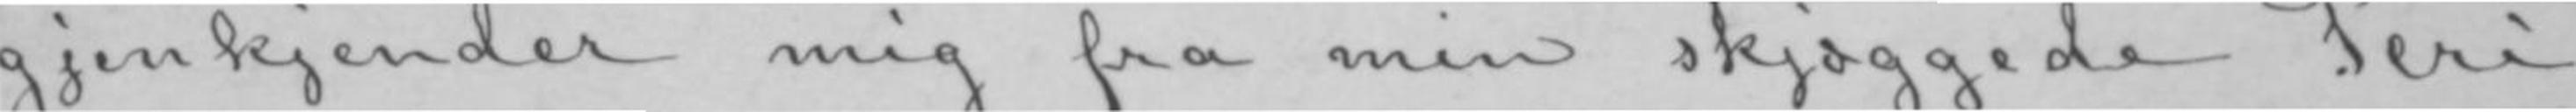gjenkjender mig fra min skjæggede Peri-
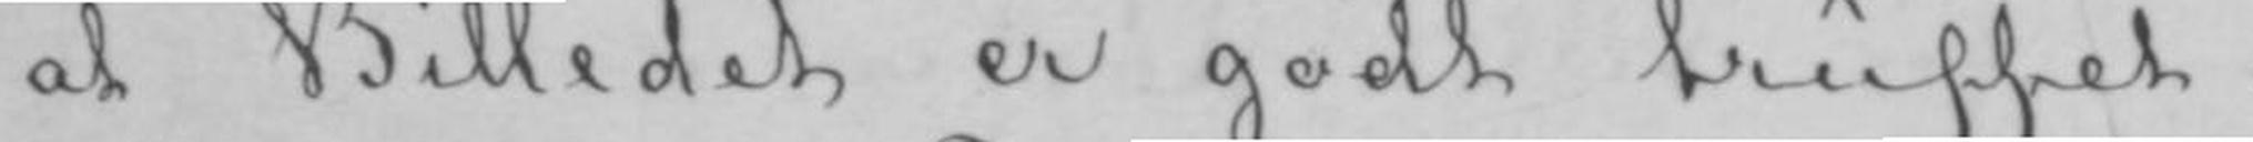at Billedet er godt truffet.
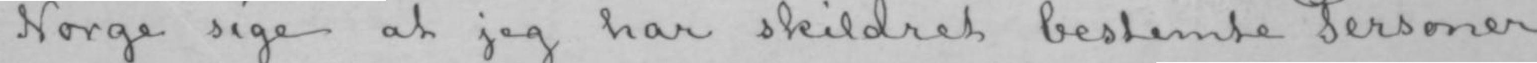Norge sige at jeg har skildret bestemte Personer
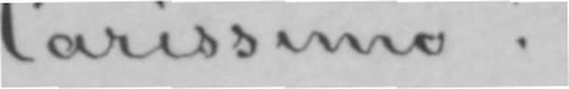Carissimo!
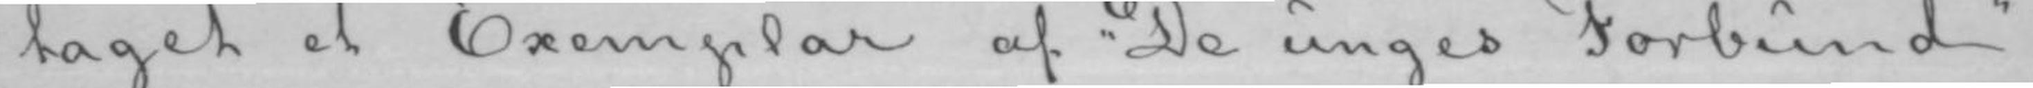taget et Exemplar af «De unges Forbund»,
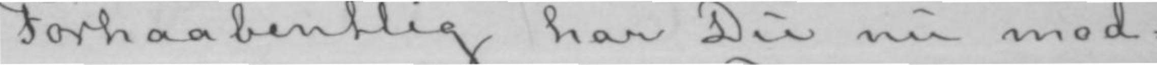Forhaabentlig har Du nu mod-
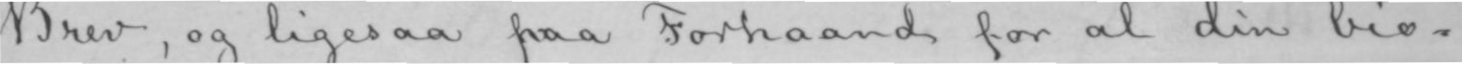Brev, og ligesaa paa Forhaand for al din bio-
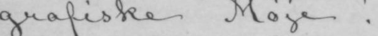grafiske Møje!
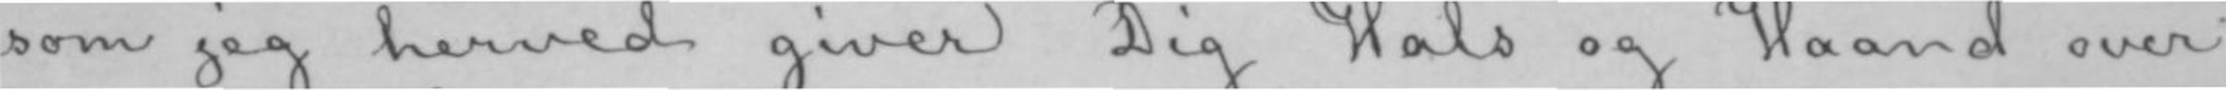som jeg herved giver Dig Hals og Haand over
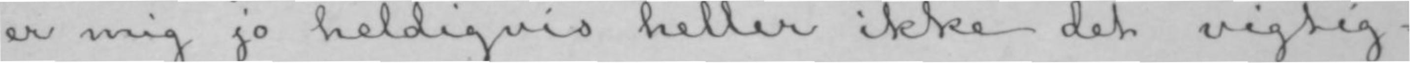er mig jo heldigvis heller ikke det vigtig-
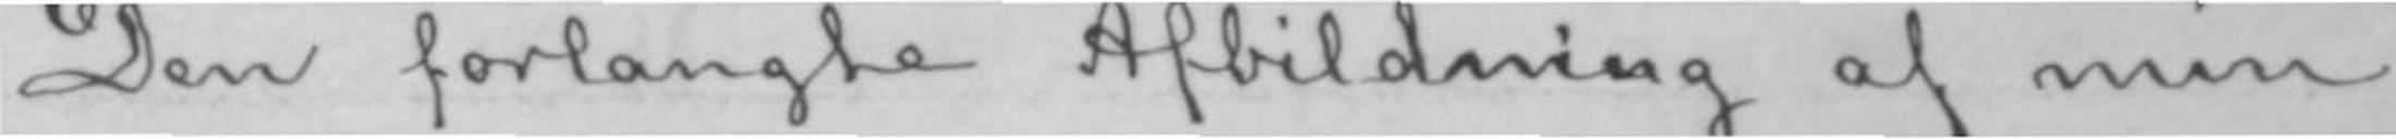Den forlangte Afbildning af min
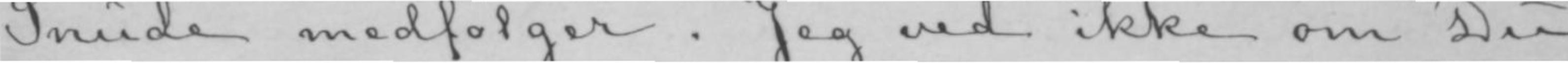Snude medfølger. Jeg ved ikke om Du
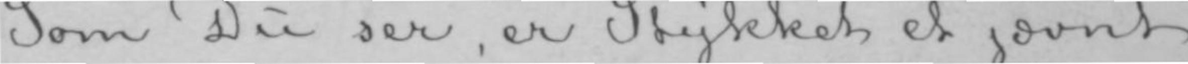Som Du ser, er Stykket et jævnt
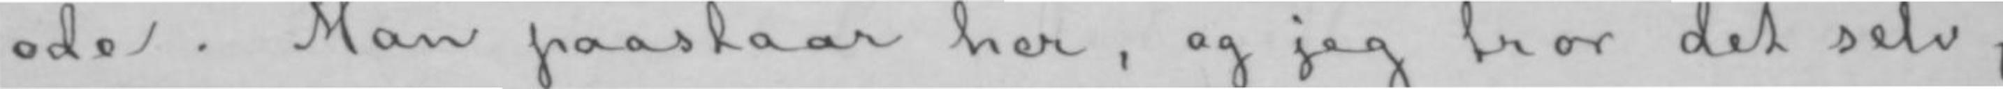ode. Man paastaar her, og jeg tror det selv,
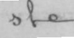ste.
